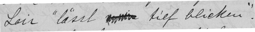Leir "läst  tief blicken".
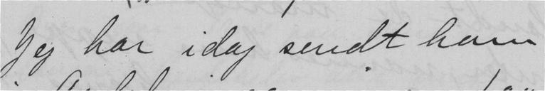Jeg har i dag sendt ham
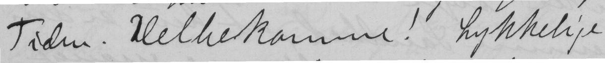Tiden. Velbekomme! Lykkelige
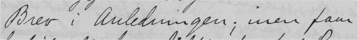Brev i Anledningen; men faar
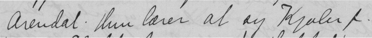Arendal. Hun lærer at sy Kjoler f.
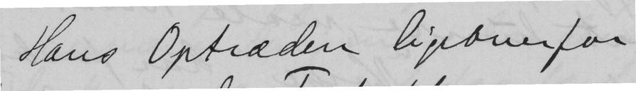Hans Optræden ligeoverfor
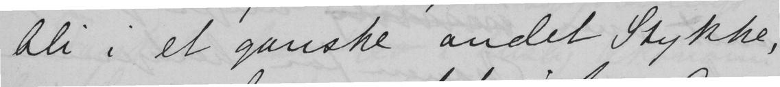bli i et ganske andet Stykke,
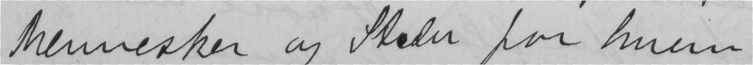Mennesker og Steder for hvem
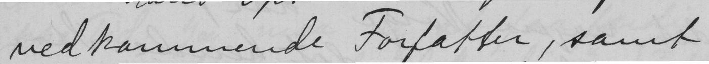vedkommende Forfatter, samt
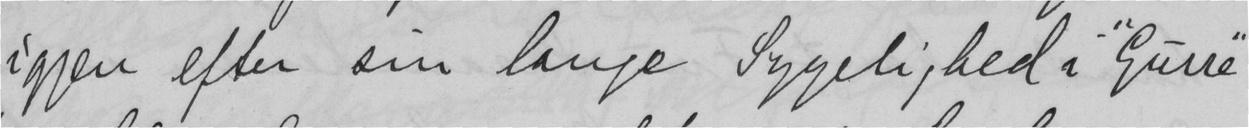igjen efter sin lange sygelighed i "Gurre"
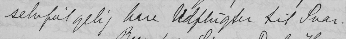selvfølgelig bare Udflugter til Svar.
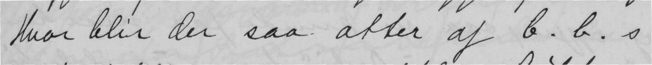Hvor blir der saa atter af b.b.s
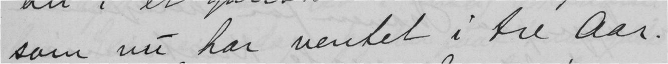som nu har ventet i tre Aar.
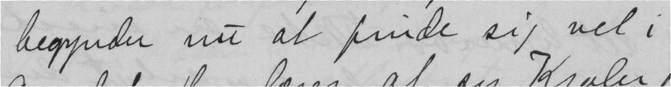begynder nu at finde sig vel i
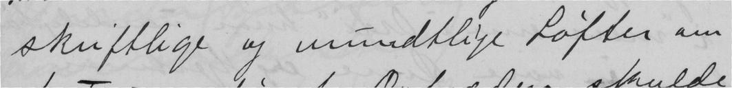skriftlige og mundtlige Løfter om
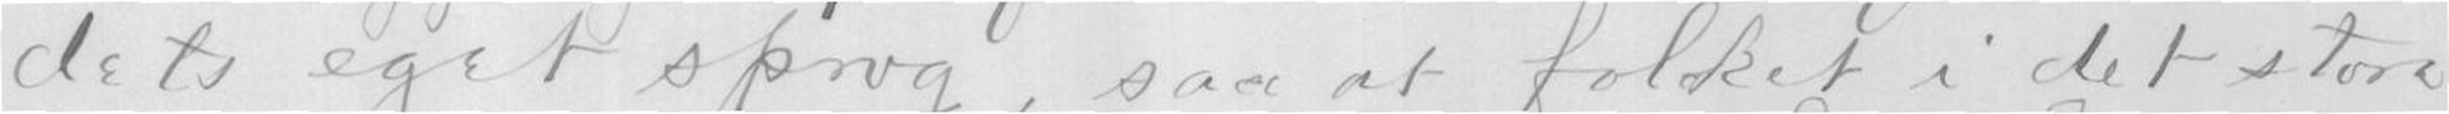dets eget sprog, saa at folket i det store
